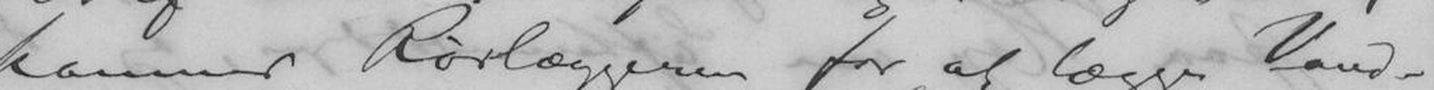kommer Rørlæggeren for at lægge Vand-
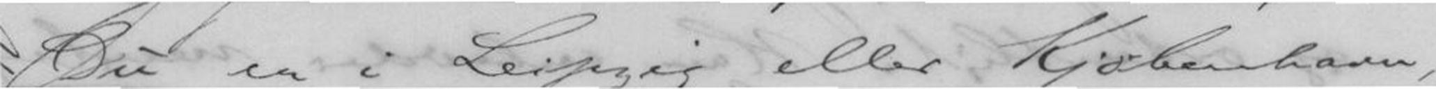Du er i Leipzig eller Kjøbenhavn,
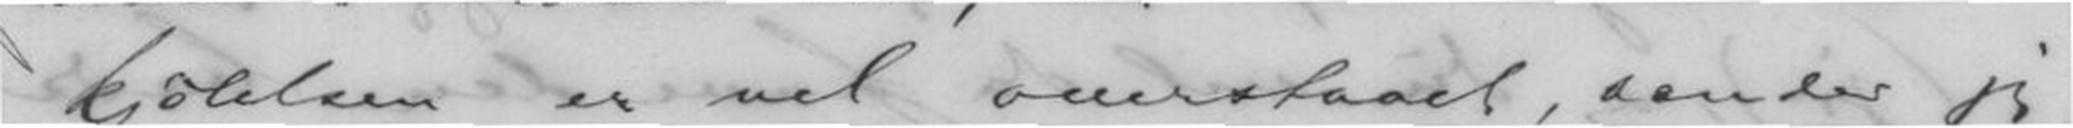kjølelsen er vel overstaaet, sender jeg
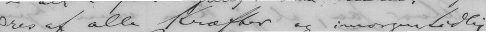res af alle Kræfter og imorgentidlig
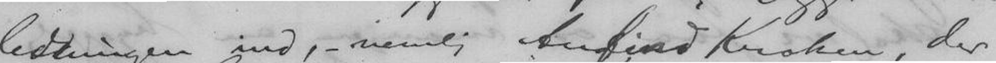ledningen ind, - nemlig Anfind Kroken, der
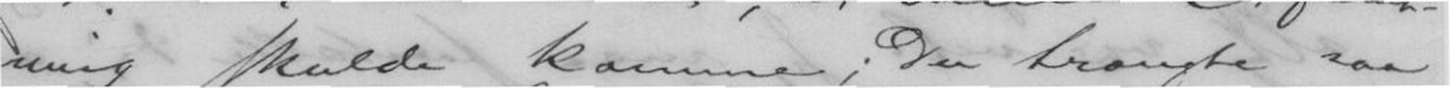ning skulde komme; Du trængte saa
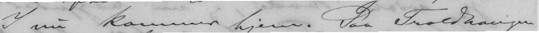I nu kommer hjem. Paa Troldhaugen
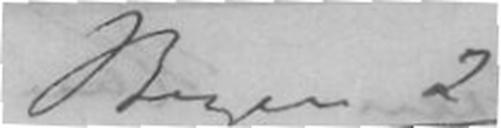Bergen 23/4 96
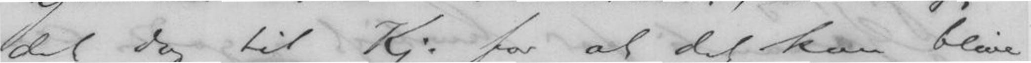det dog til Kj. for at det kan blive
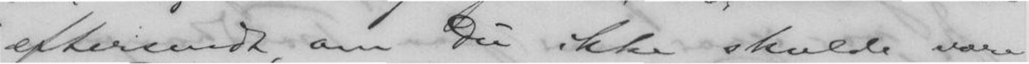eftersendt, om Du ikke skulde være
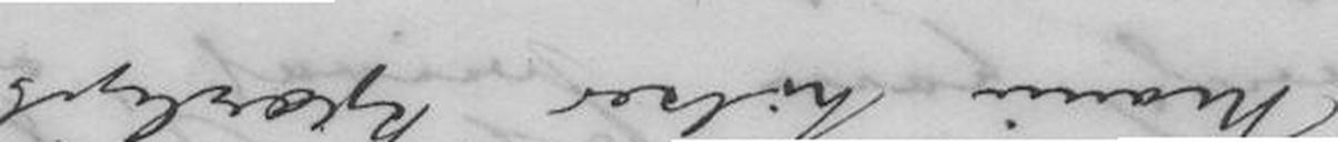Marie hilser kjærligst!
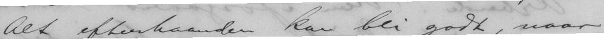Alt efterhaanden kan bli godt, naar
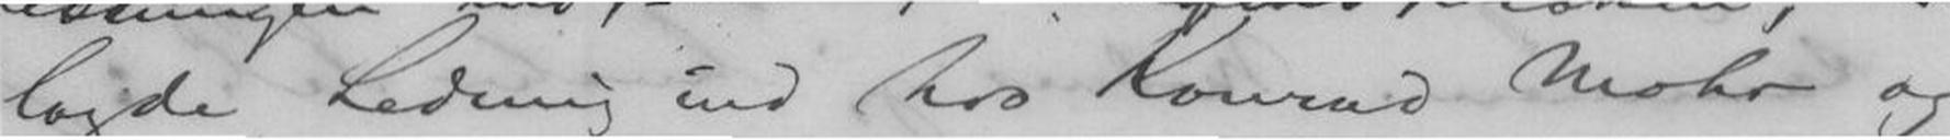lagde Ledning ind hos Konrad Mohr og
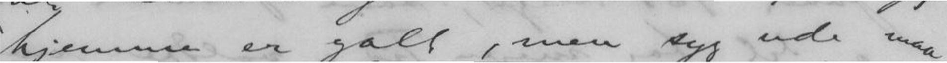hjemme er galt, men syg ude maa
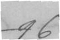Skrevet oppned øverst på siden:
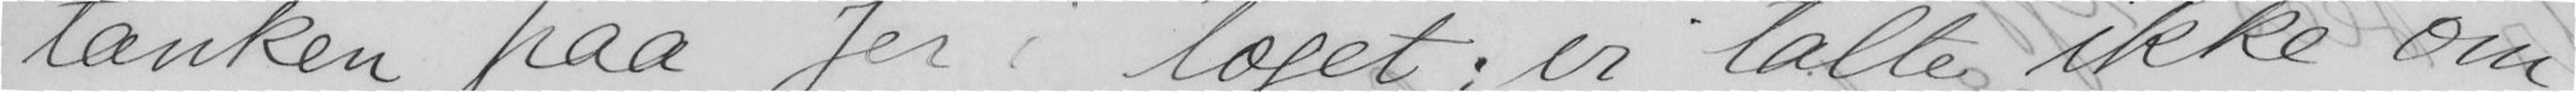tanken paa Jer i toget; vi talte ikke om
